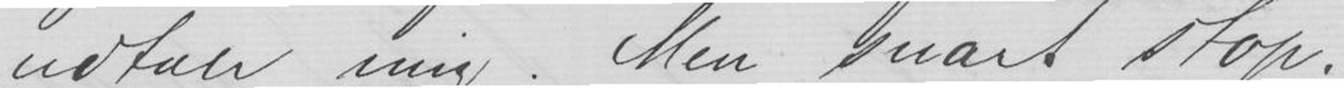udtale mig. Men snart stop-
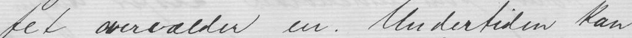fet overvælder en. Undertiden kan
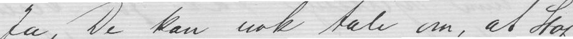Ja De kan nok tale om, at Stof-
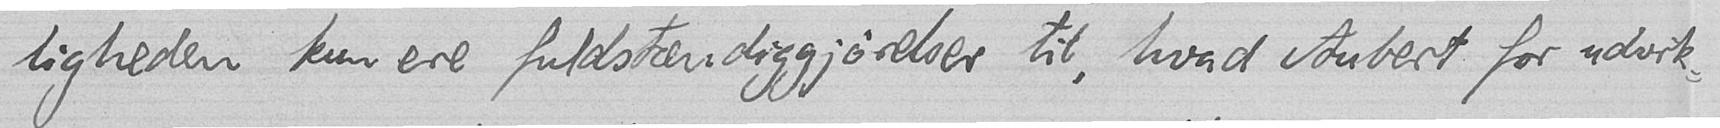ligheden kun ere fuldstændiggjørelser til, hvad Aubert for udvik-
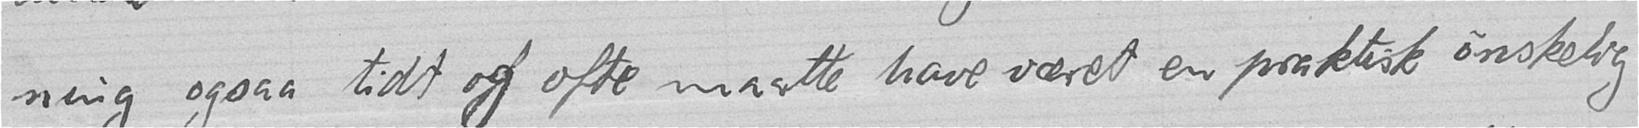ning ogsaa tidt og ofte maatte have været en praktisk ønskelig
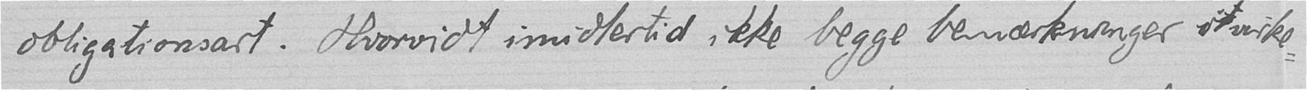obligationsart. Hvorvidt imidlertid ikke begge bemærkninger i virke-
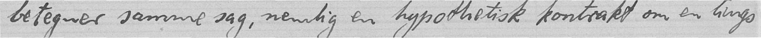betegner samme sag, nemlig en hypothetisk kontrakt om en tings
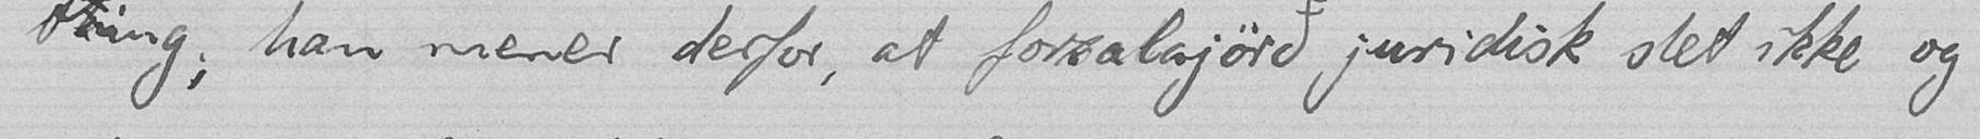ting; han mener derfor, at forsölujörð juridisk slet ikke og
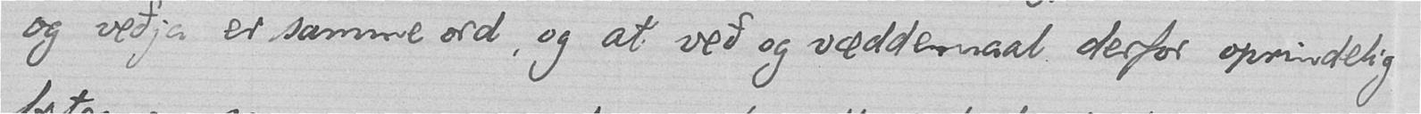og veðja er samme ord, og at veð og væddemaal derfor oprindelig
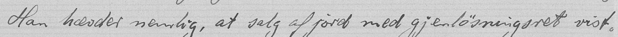Han hævder nemlig, at salg af jord med gjenløsningsret vist-
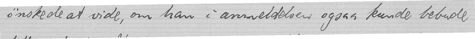ønskede at vide, om han i anmeldelsen ogsaa kunde bebude
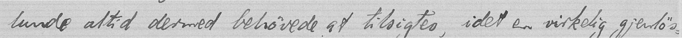lunde altid dermed behøvede at tilsigtes, idet en virkelig gjenløs-
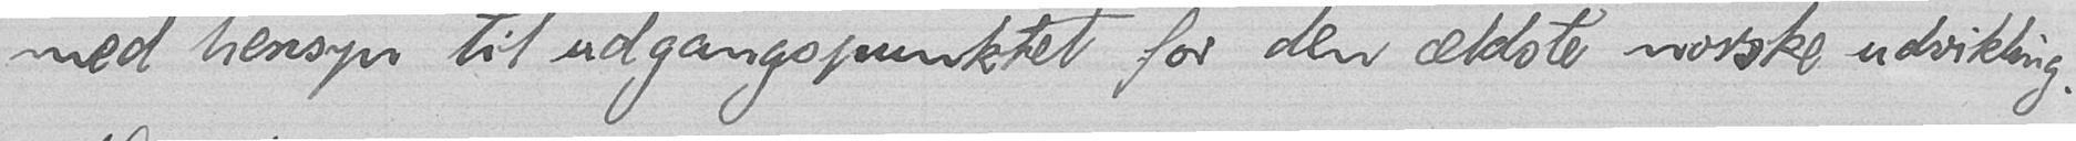med hensyn til udgangspunktet for den ældste norske udvikling.
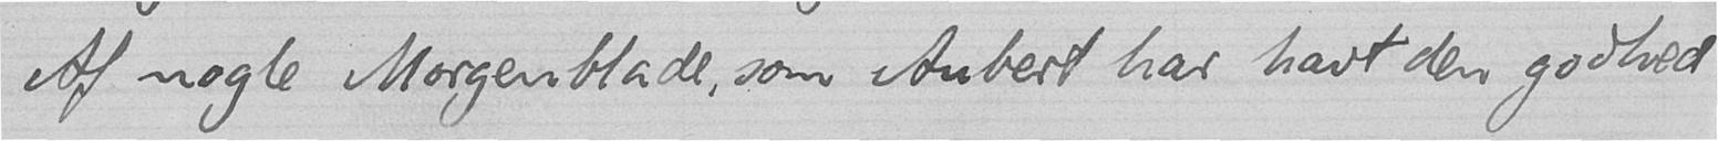Af nogle Morgenblade, som Aubert har havt den godhed
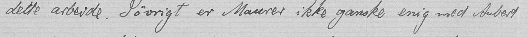dette arbeide. Iøvrigt er Maurer ikke ganske enig med Aubert
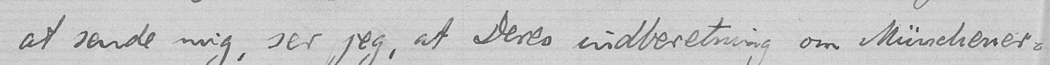at sende mig, ser jeg, at Deres indberetning om Münchener-
